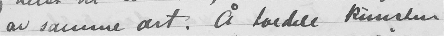av samme art. Å tvedele kunsten
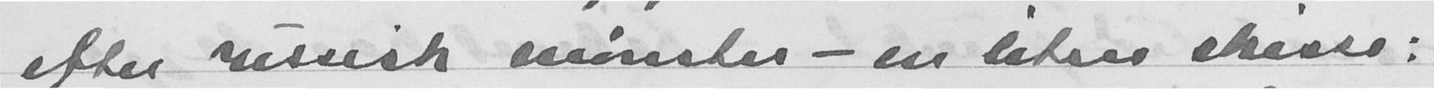efter russisk mønster - en liten skisse:
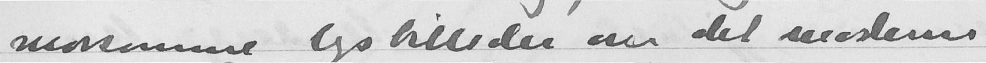morsomme lysbilleder om det snøderns
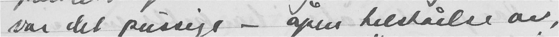var det pussige - åpen tilståelse av,
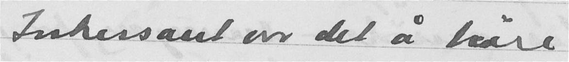Interessant var det å høre
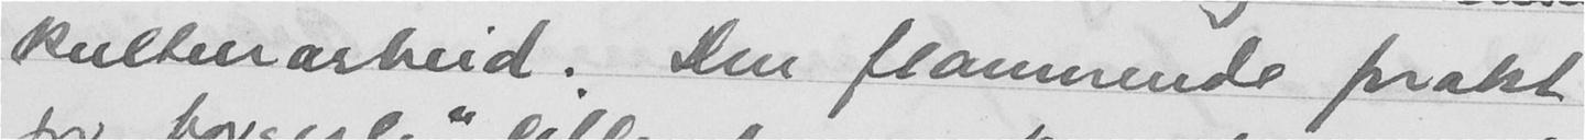kulturarbeid. Den flammende forakt
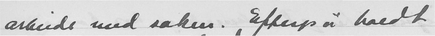arbeide med saken. Efterpå holdt
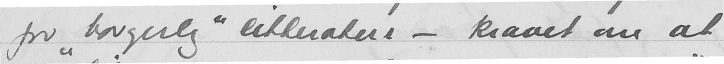for "borgerlig" litteratur - kravet om at
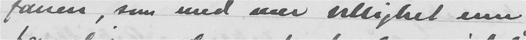fanen, som med mer villighet en
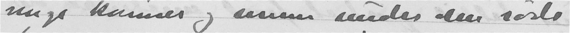unge kvinner og menn under den røde
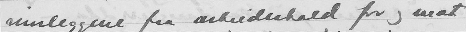innleggene fra arbeiderhold for og mot
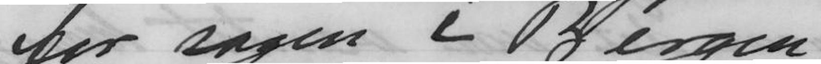for sagen i Bergen
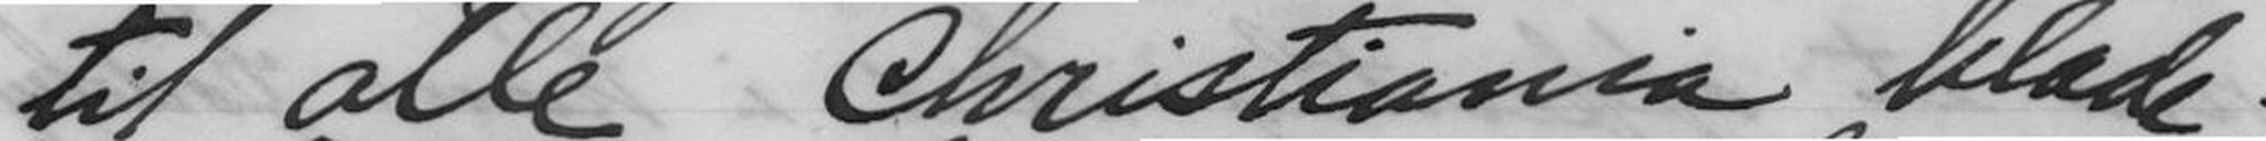til alle Christiania blade;
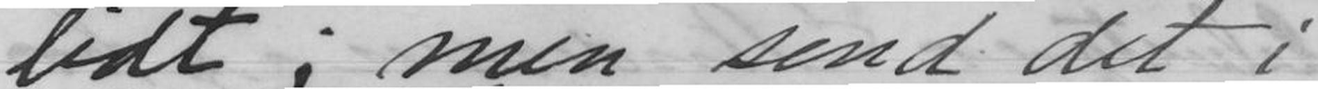lidt; men send det i
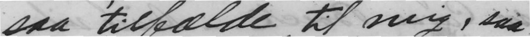saa tilfælde til mig, saa
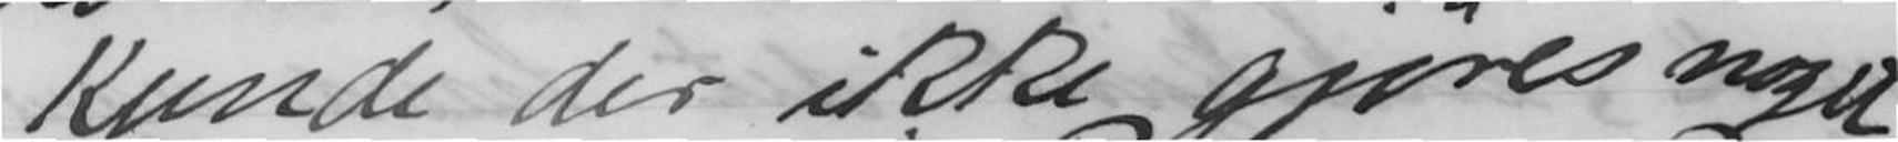Kunde der ikke gjøre noget
